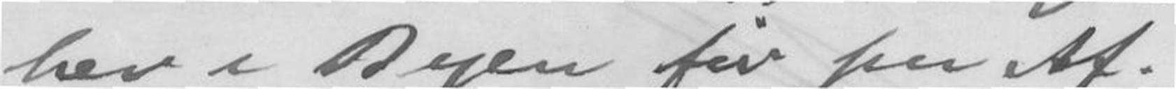her i Byen før sin Af-
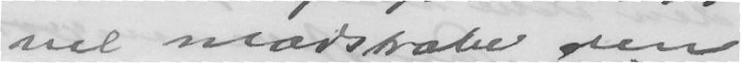vil modstræbe den
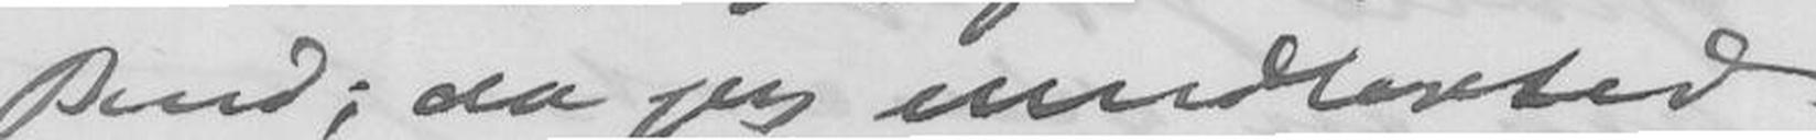Bind; da jeg midlertid
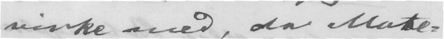virke med, da Mate-
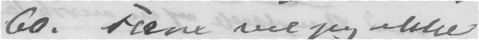60. Flere vil jeg ikke
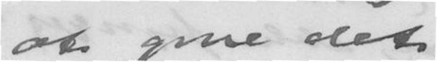at give det
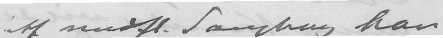Af medfl. Sangbog har
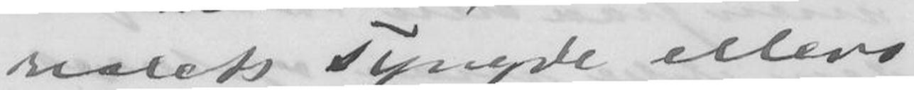rialets Tyngde ellers
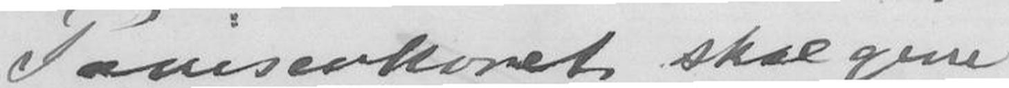Pariserkoret skal give
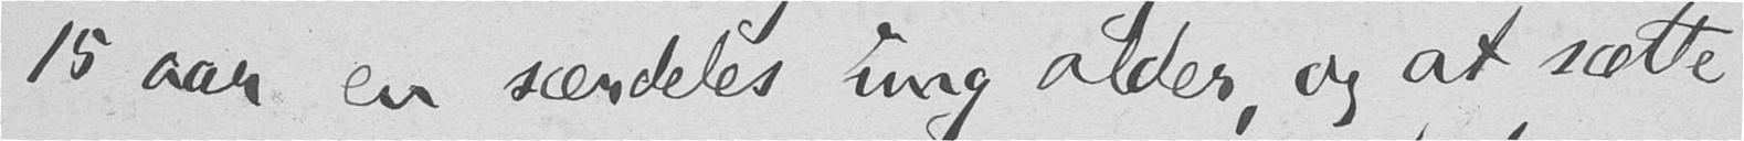15 aar en særdeles ung alder, og at sætte
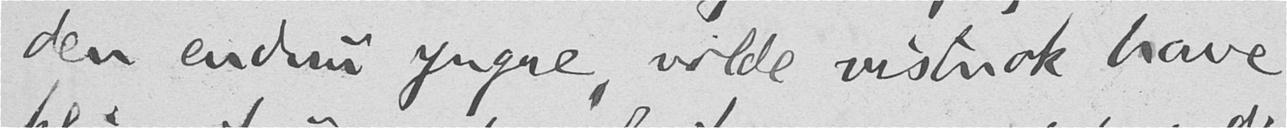den endnu yngre, vilde vistnok have
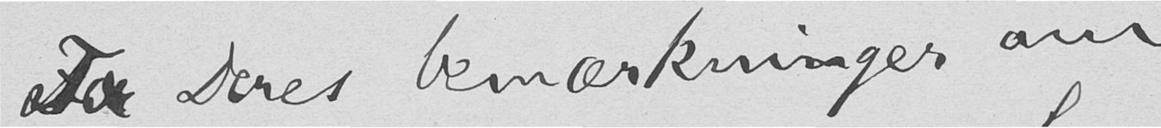For Deres bemærkninger om
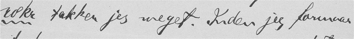rækr takker jeg meget. Inden jeg formaar
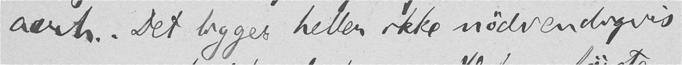aarh.. Det ligger heller ikke nødvendigvis
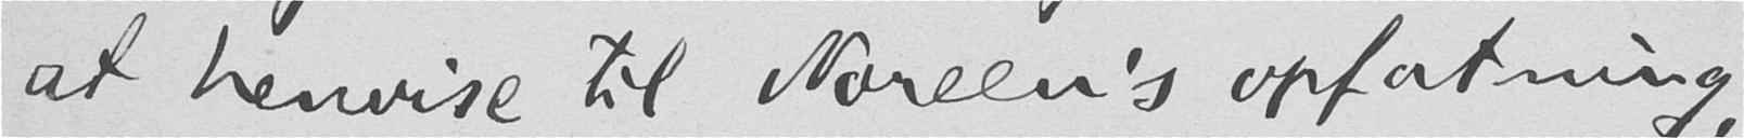at henvise til Noreen's opfatning,
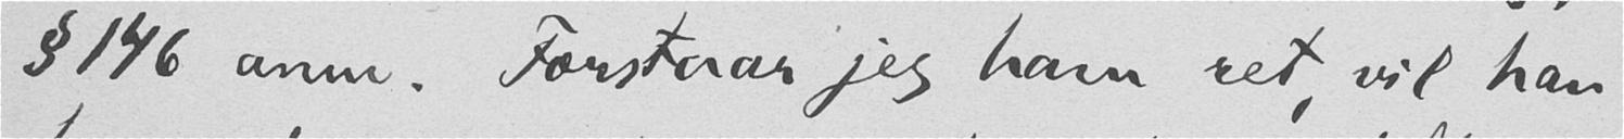§146 anm. Forstaar jeg ham ret, vil han
